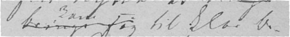til klar Be-
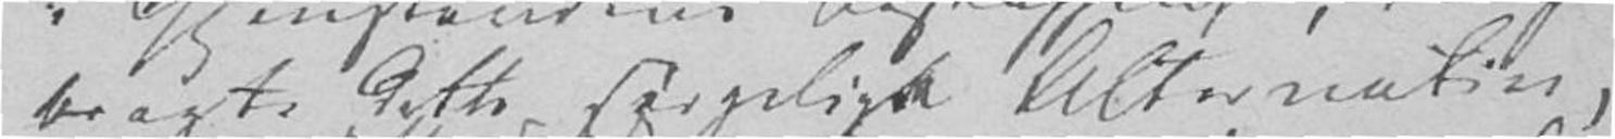bragte dette sørgelige Alternativ,
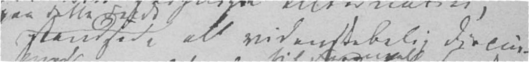standsede all videnskabelig Discus-
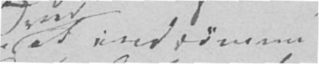at indrømme
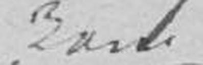komme
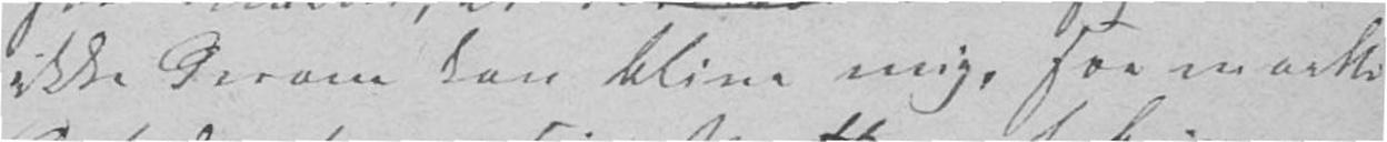ikke derom kan blive enig, saa maatte
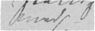med
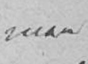man
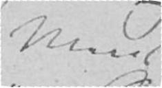Men
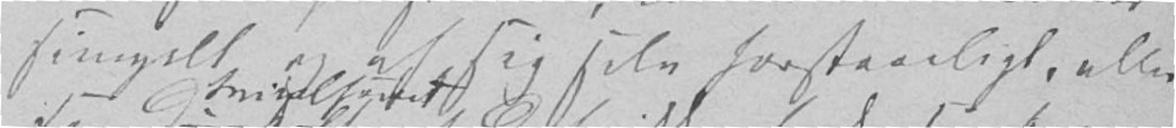simpelt og af sig forstaaeligt, eller
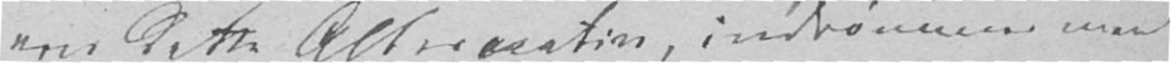om dette Alternativ, indrømmer man
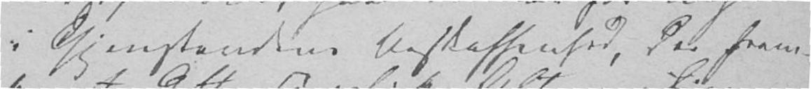i Gjenstandens Beskaffenhed, der fram-
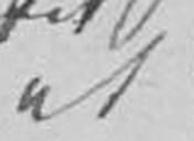at
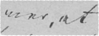mer, at
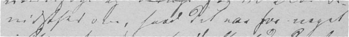vidsthed om, hvad det var for noget
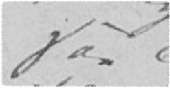saa
